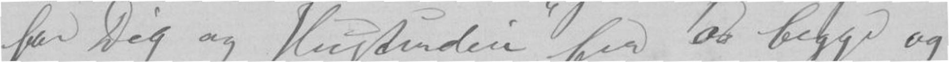for Dig og Hustruden din fra os begge og
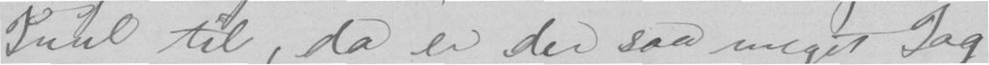Juul til, da er der saa meget Jag,
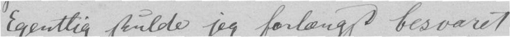Egentlig skulde jeg forlængst besvaret
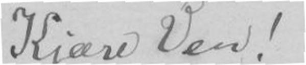Kjære Ven!
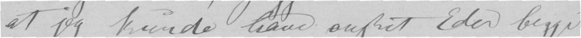at jeg kunde have ønsket Eder begge
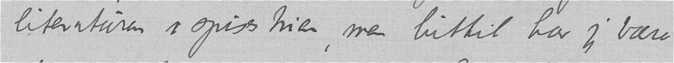literaturen i spisestuen, men hittil har jeg bare
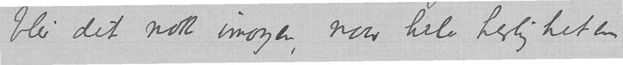blir det nok imorgen, naar hele herligheten
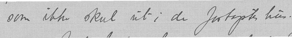som ikke skal ut i de fortaptes hus.
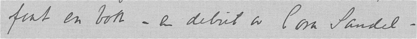faat en bok – en debut av Cora Sandel –
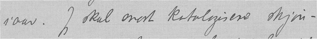iaar. Jeg skal snart katologisere skjøn-
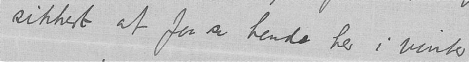sikkert at faa se hende her i vinter
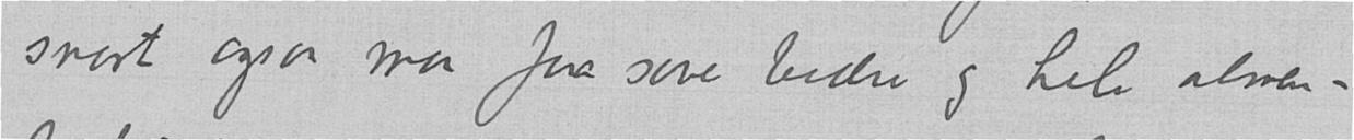snart ogsaa maa faa sove bedre og hele almen –
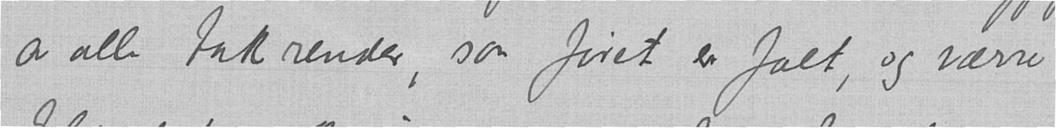av alle takrender, saa føret er fælt, og værre
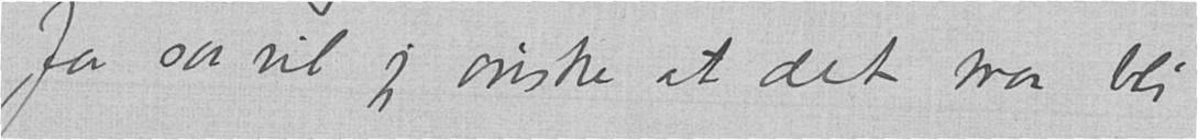Ja saa vil jeg ønske at det maa bli
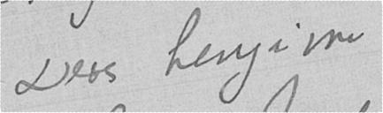Deres hengivne
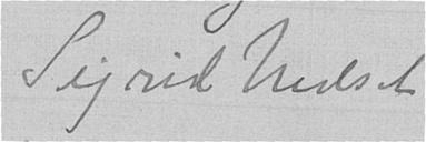Sigrid Undset
